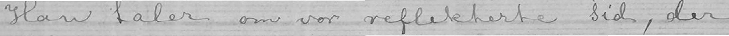Han taler om vor reflekterte Tid, der
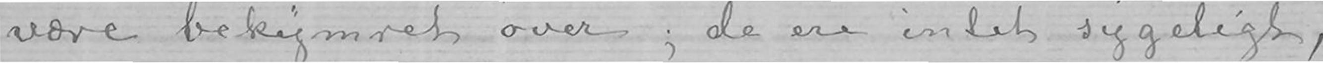være bekymret over; de ere intet sygeligt,
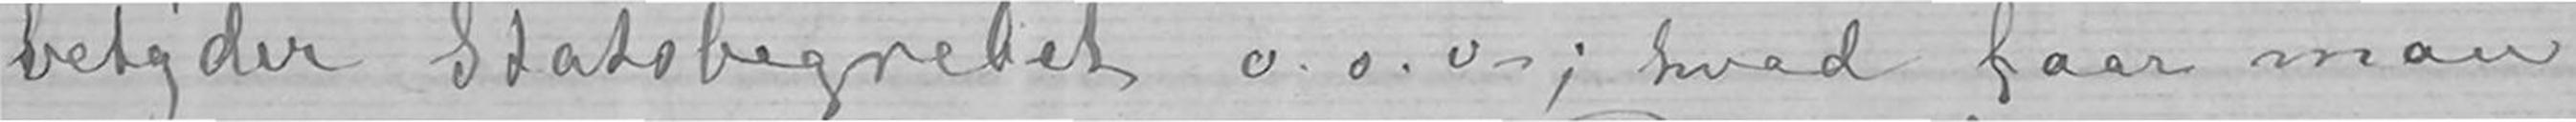betyder Statsbegrebet o. s. v.; hvad faar man
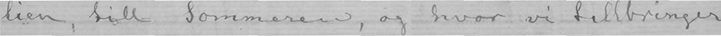lien, till Sommeren, og hvor vi tillbringer
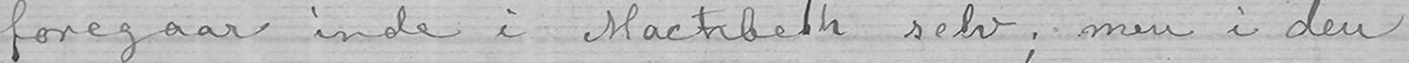foregaar inde i MacbethMach selv; men i den
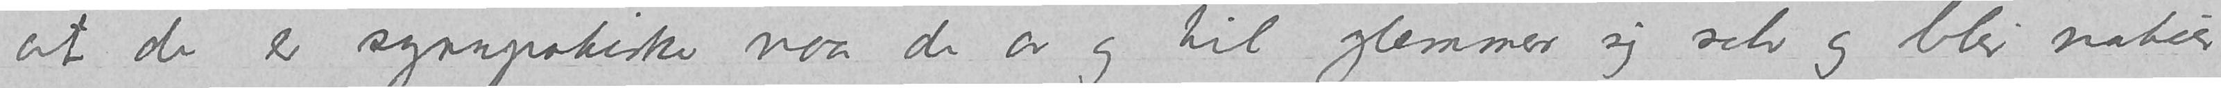at de er sympatiske naar de av og til glemmer sig selv og blir natur
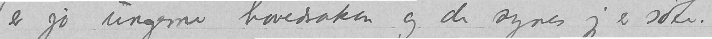er jo ungerne hovedsaken og de synes jeg er søte.
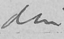din
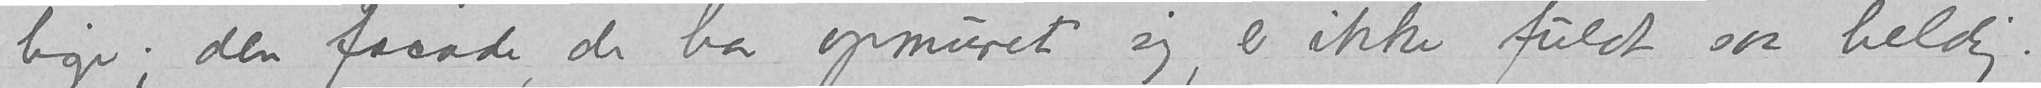lige; den fasade, de har opmuret sig, er ikke fuldt saa heldig.
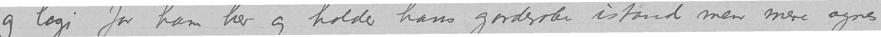og logi for ham her og holder hans garderobe istand men mere synes
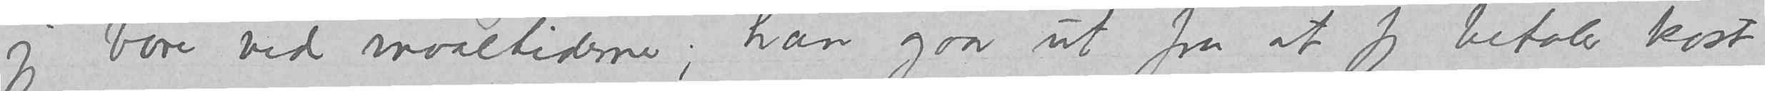jeg bare ved maaltiderne; han gaar ut fra jeg betaler kost
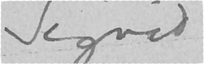Sigrid
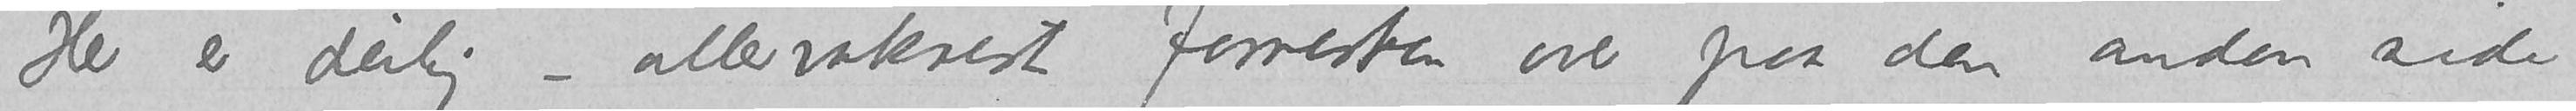Her er deilig – allervakrest forresten over paa den anden side
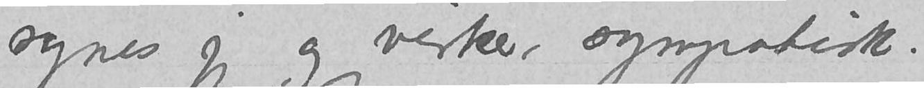synes jeg og virker sympatisk
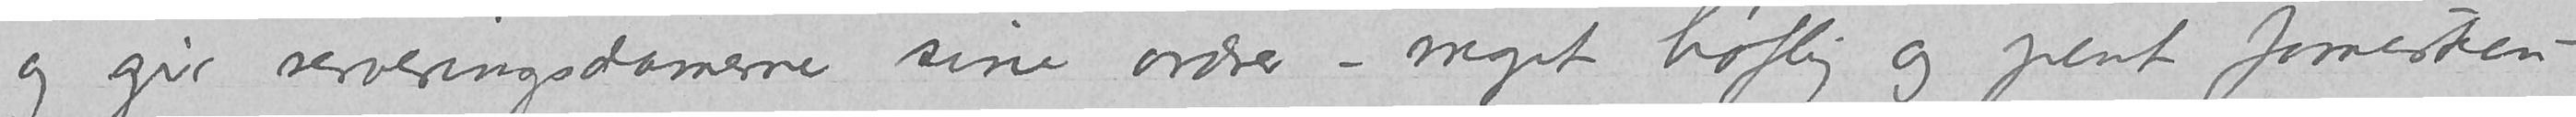og gir serveringsdamerne sine ordrer – meget høflig og pent forresten –
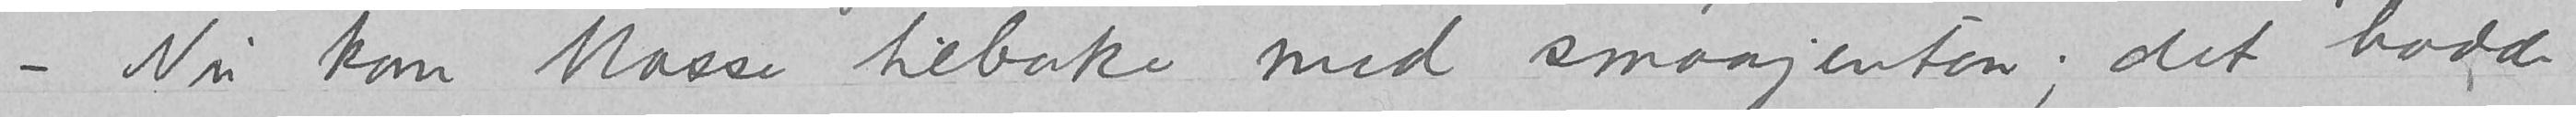Nu kom Mosse tilbake med smaajenten; det hadde
